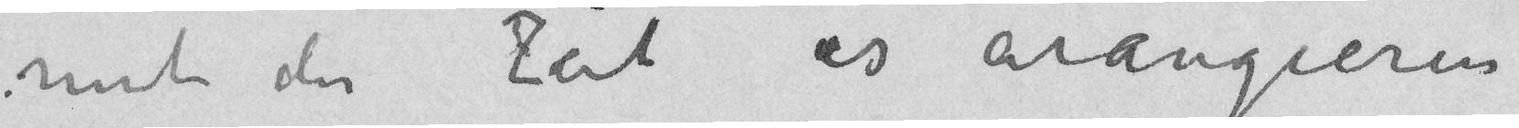mit der Zeit es arangieren
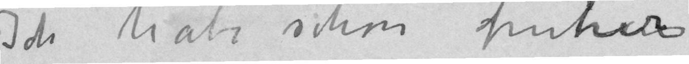Ich habe schon fruher
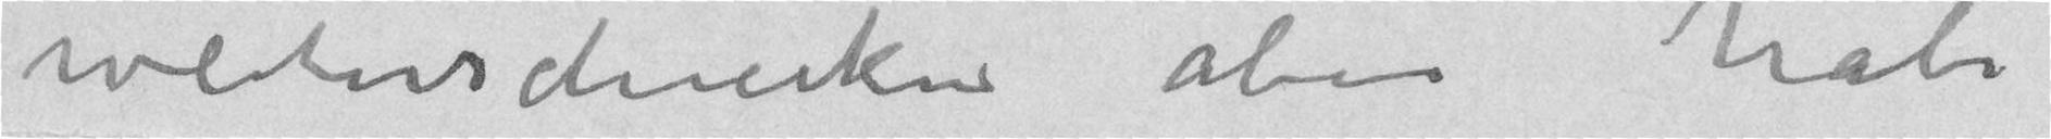weitersdrucken aber habe
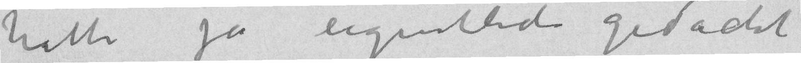hatte ja eigentlich gedacht
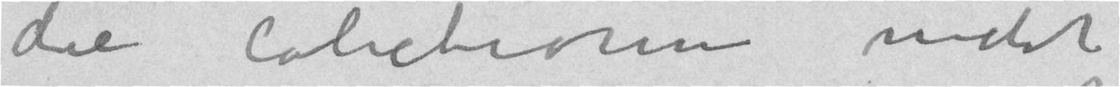die Colectionen nicht
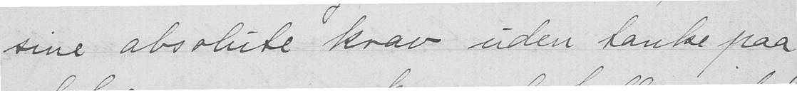sine absolute krav uden tanke paa
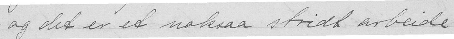- og det er et noksaa stridt arbeide
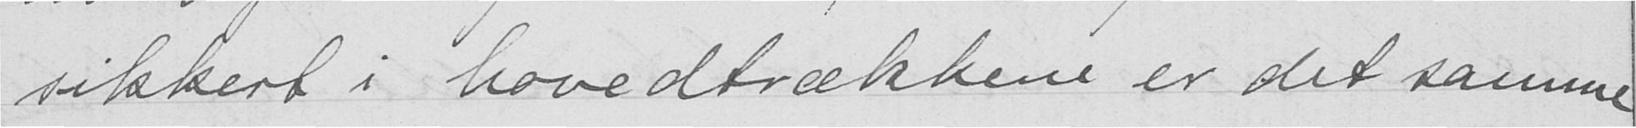sikkert i hovedtrækkene er det samme
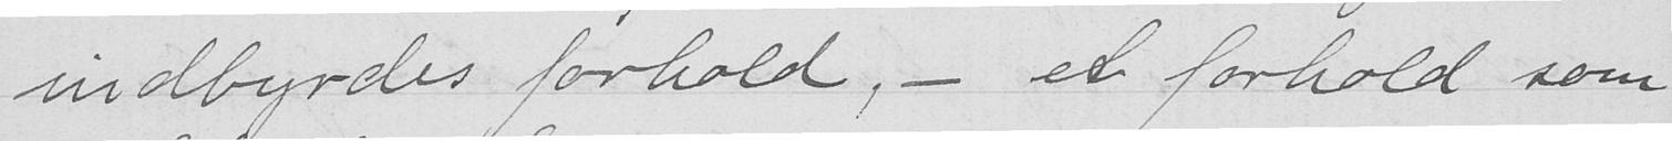indbyrdes forhold, - et forhold som
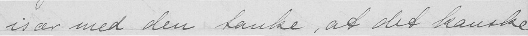især med den tanke, at det kanske
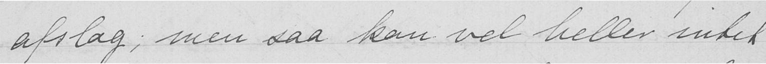afslag; men saa kan vel heller intet
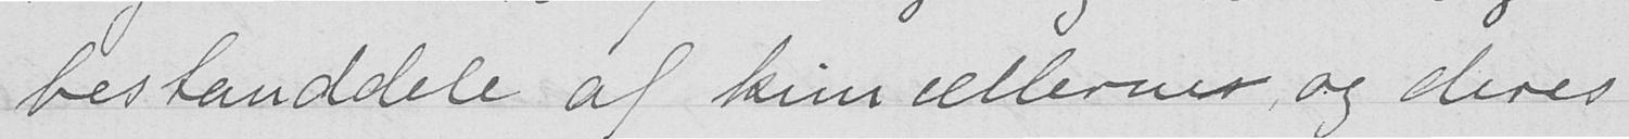bestanddele af kun cellerner, og deres
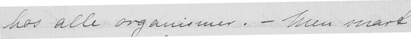hos alle organismer. - Men snart
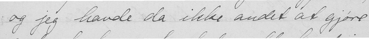og jeg havde da ikke andet at gjøre
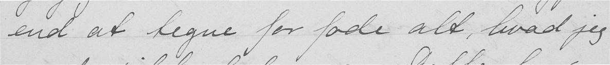end at tegne for fode alt, hvad jeg
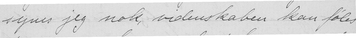synes jeg nok, videnskaben kan føles
